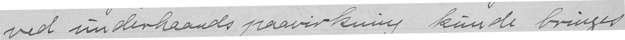ved underhaands paavirkning kunde bringes
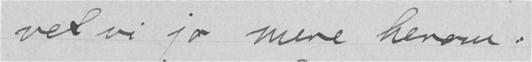ved vi jo mere herom.
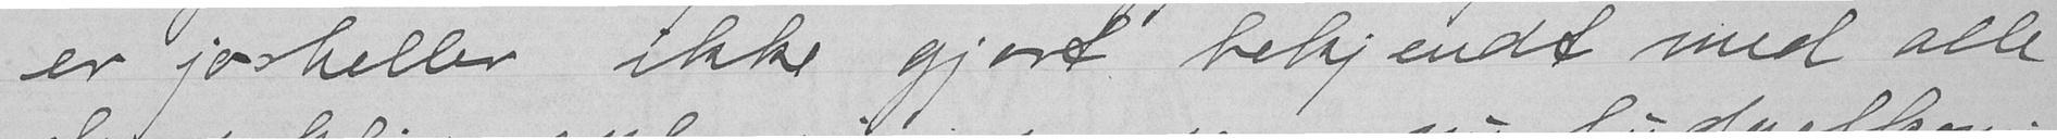er jo heller ikke gjort bekjendt med alle
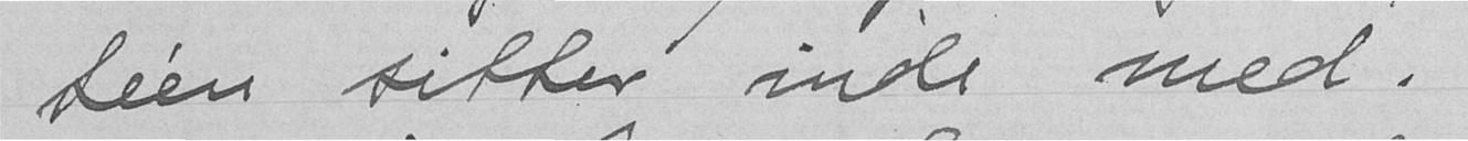teen sitter inde med.
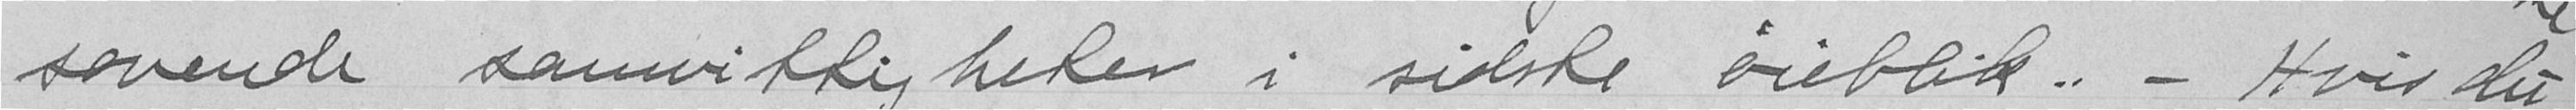sovende samvitligheder i sidste øieblik. - Hvis du
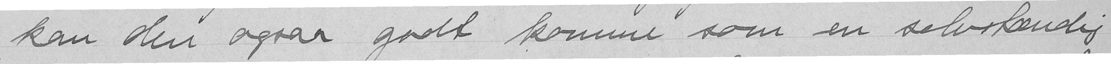kan den ogsaa godt komme som en selvstændig
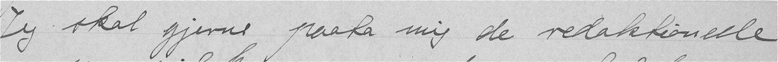Jeg skal gjerne paata mig de redaktionelle
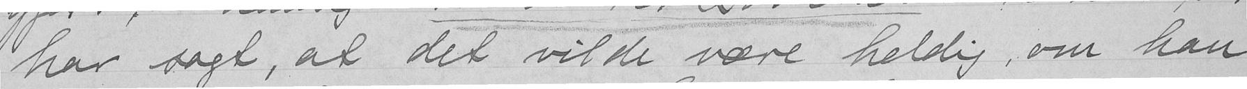har sagt, at det vilde være heldig, om han
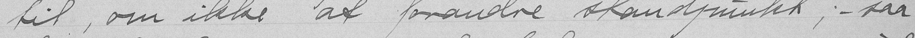til, om ikke at forandre standpunkt, - saa
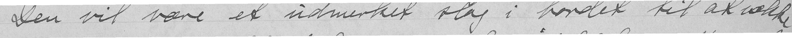Den vil være et udmærket slag i bordet til at vække
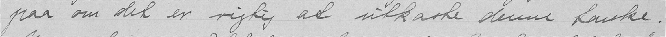paa om det er rigtig at utkaste denne tanke.
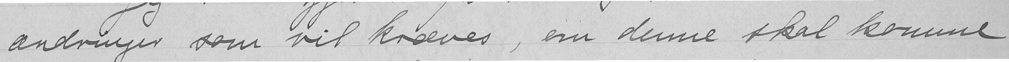ændringer som vil kræves, om denne skal komme
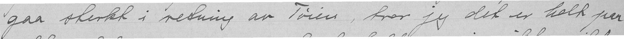gaa sterkt i retning av Tøien, tror jeg det er helt paa
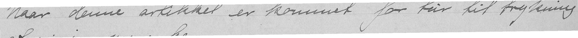Naar denne artikkel er kommet for tur til trykning
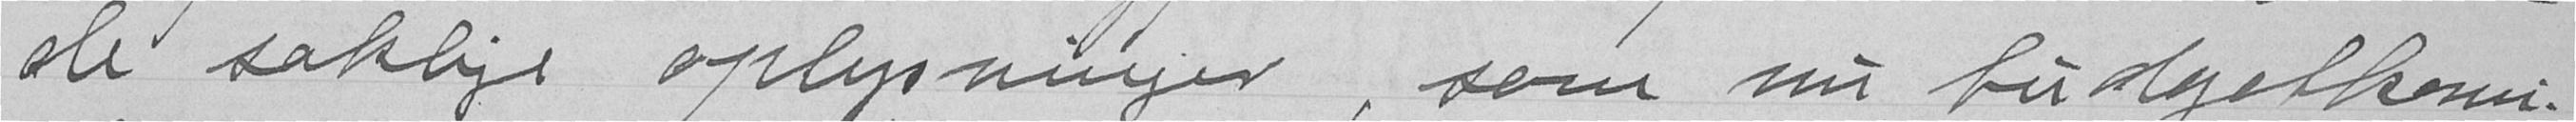de saklige oplysninger, som nu budgetkomi-
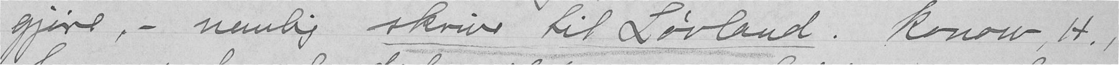gjøre - nemlig skrive til Løvland. Konow, H.,
